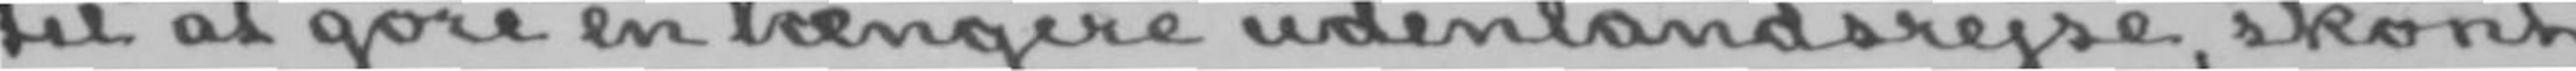til at gøre en længere udenlandsrejse, skønt
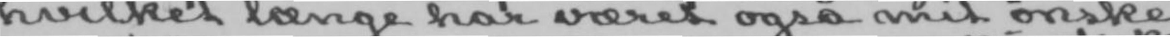hvilket længe har været også mit ønske.
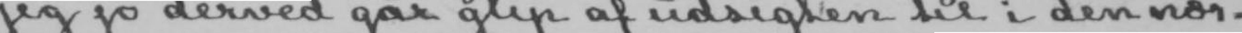jeg jo derved går glip af udsigten til i den nær-
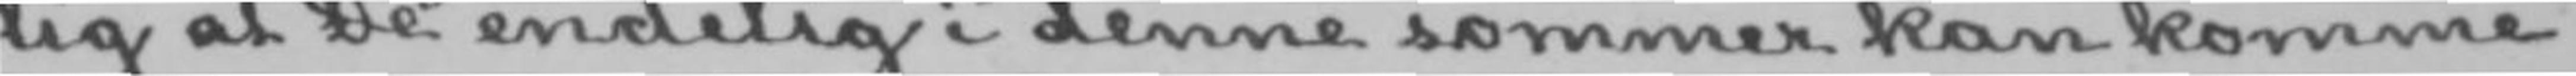lig at De endelig i denne sommer kan komme
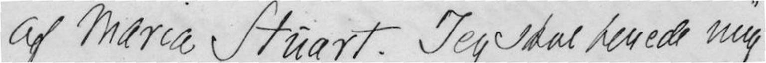af Maria Stuart. Jeg skal berede mig
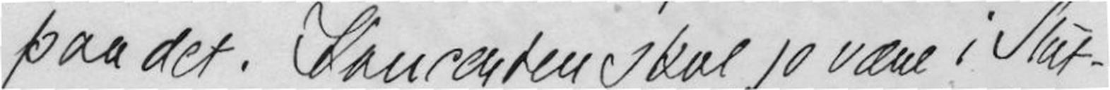paa det. Koncerten skal jo være i Slut-
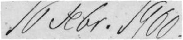10 Febr. 1900
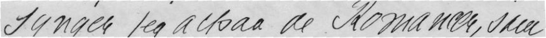synger jeg altsaa de Romancer, som
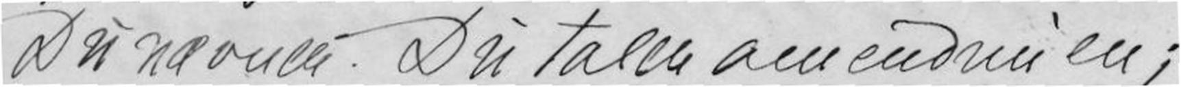Du nævnte. Du taler om endnu en;
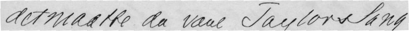det maatte da være Taylors Sang
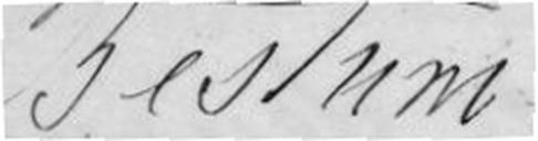Bestum
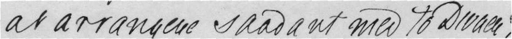at arrangere saadant med to "Divalle",
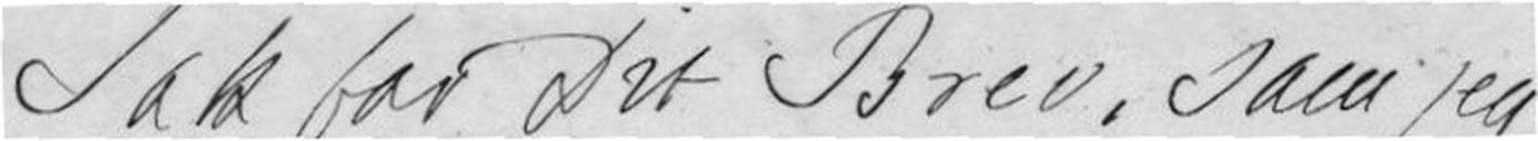Tak for dit Brev, som jeg
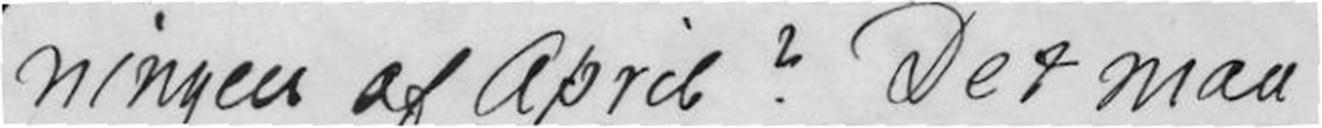ningen af April? Det maa
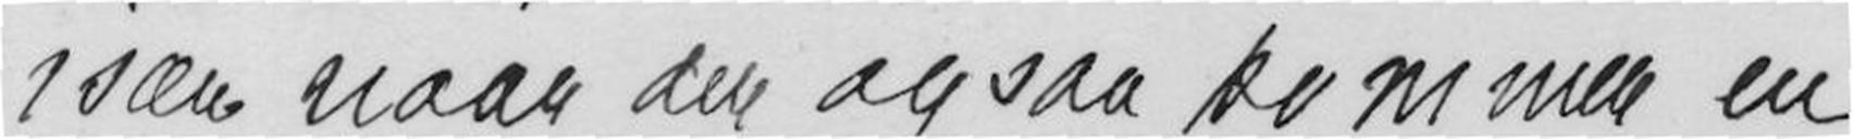især naar der ogsaa kommer en
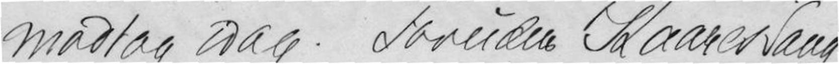modtog idag. Foruden Kaaret Sang
